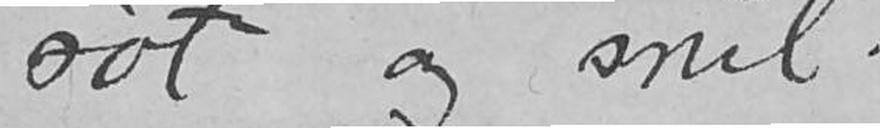søt og snil.
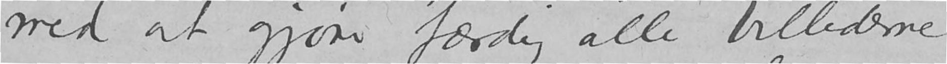med at gjøre færdig alle billederne
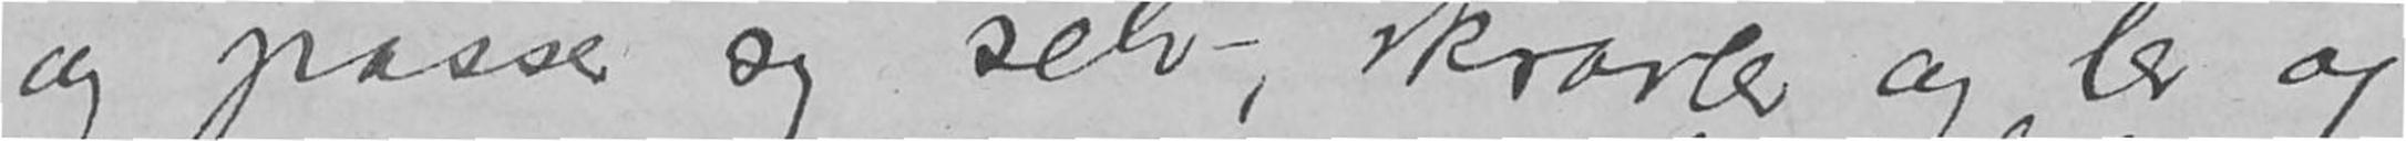og passer sig selv, skravler og ler og
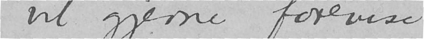nu, at jeg vil gjerne forevise
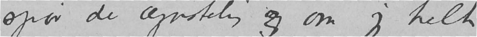spør de ængstelig  om jeg helt
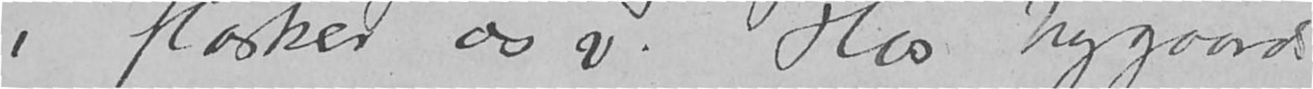i flasker osv. Hos Nygaard
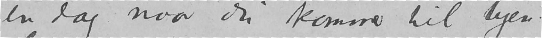en dag naar du kommer til byen?
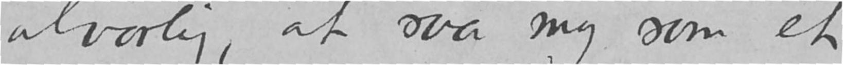alvorlig, at saa my som et
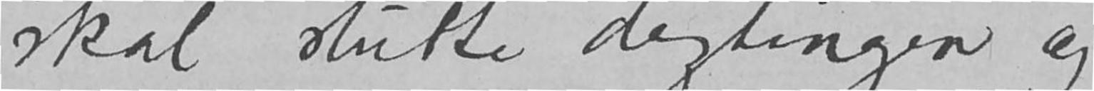skal slutte digtingen og
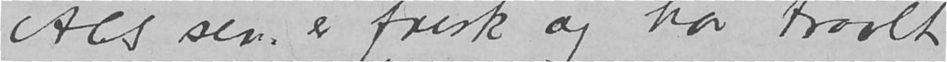Als sen er frisk og har travlt
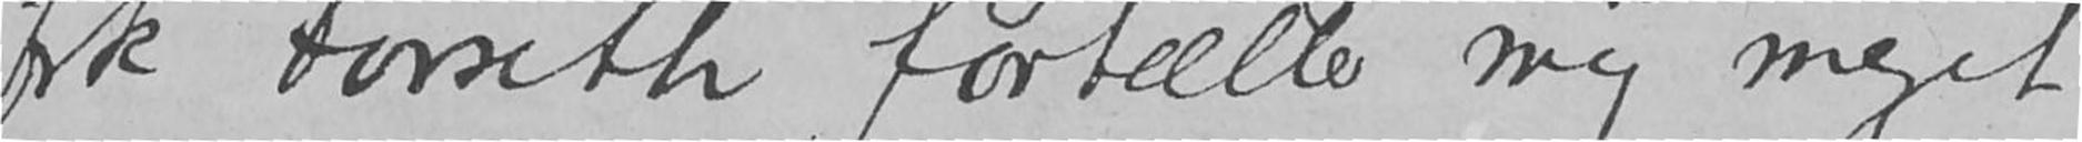frk Forseth fortæller mig meget
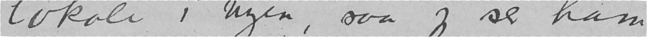lokale i byen, saa jeg ser ham
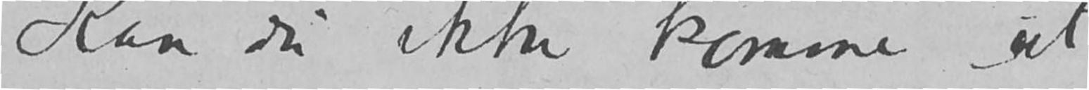Kan du ikke komme ut
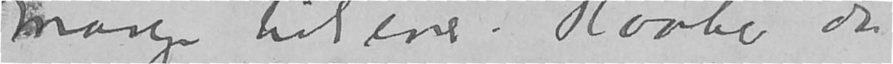Mange hilsener. Haaber du
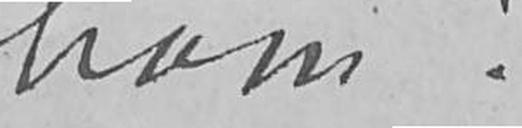ham!
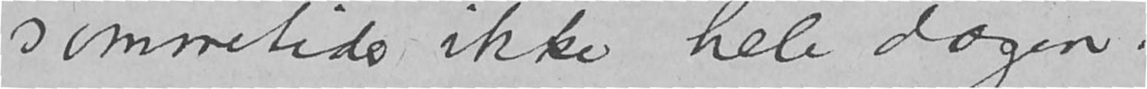sommetider ikke hele dagen.
